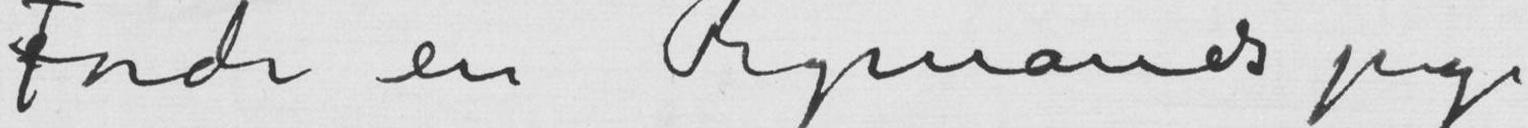Fordi en Rigmands pige
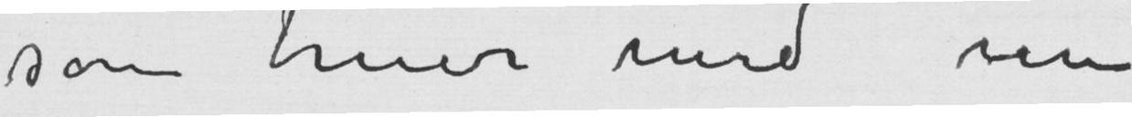som truer med en
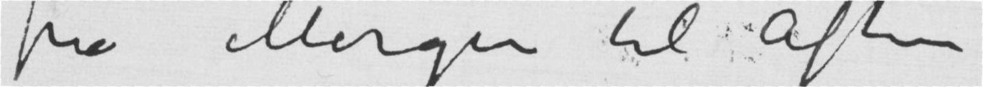fra Morgen til Aften
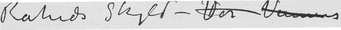Råheds Skyld – V Venn
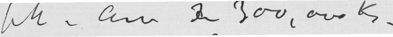fik i Arv en 300,000 Kr –
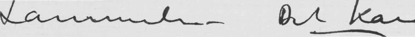Lammelse – Det kan
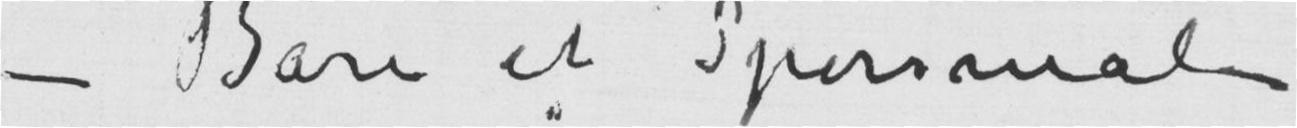– Bare et Spørsmål
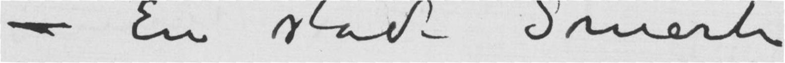– En stadi Smerte
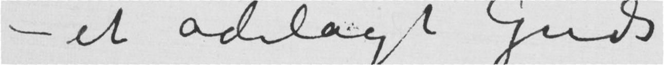– et ødelagt Guds
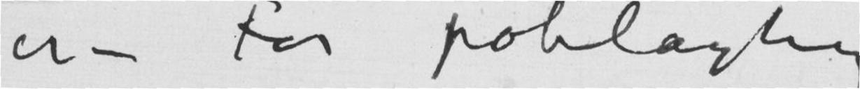er – For pøbelagtig
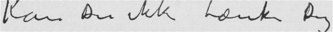Kan du ikke tænke Dig
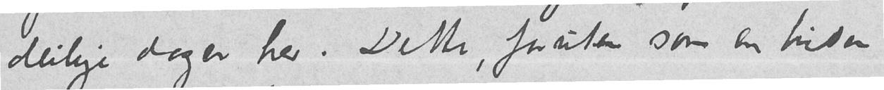deilige dager her.  Dette, foruten som en hilsen
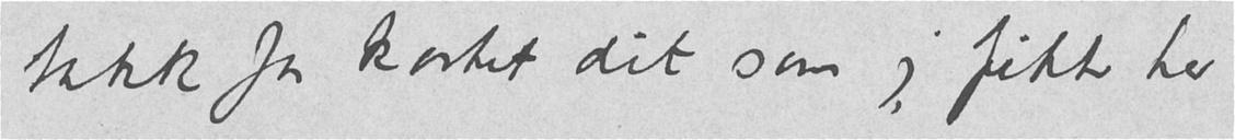takk for kortet dit som jeg fikk her
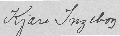Kjære Ingeborg
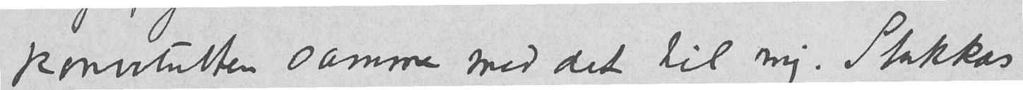konvolutten sammen med det til mig.  Stakkars
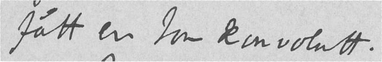fått en tom konvolutt.
